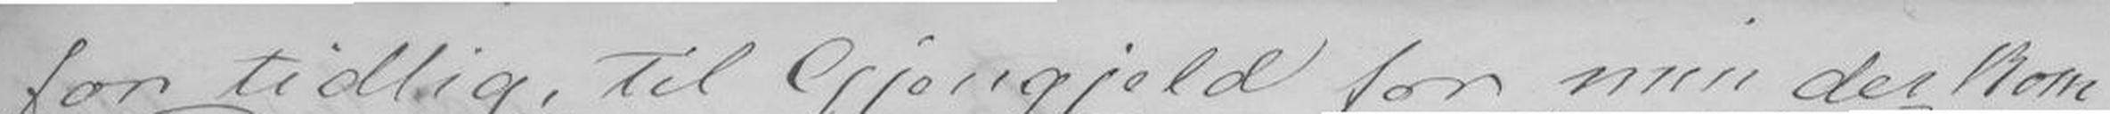for tidlig, til Gjengjeld for min der kom
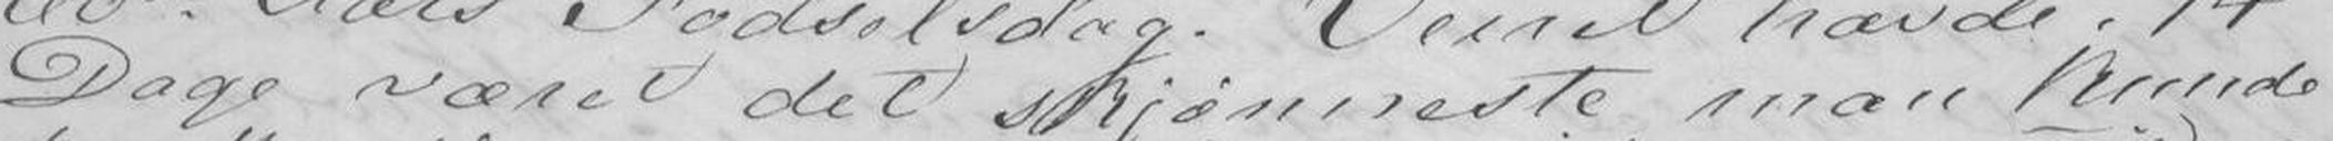Dage været det skjønneste man kunde
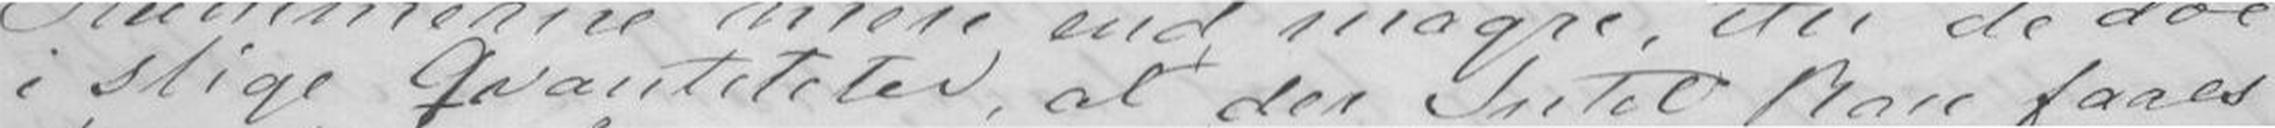i slige Qvanteteter, at der Intet kan faaes
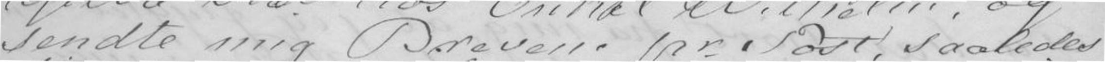sendte mig Brevene pr. Post, saaledes
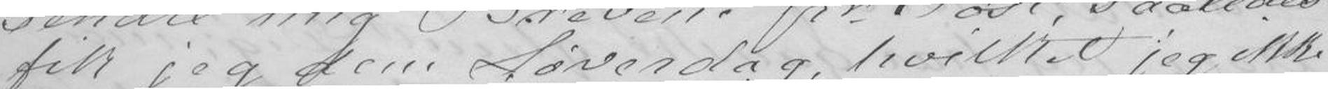fik jeg dem Løverdag, hvilket jeg ikke
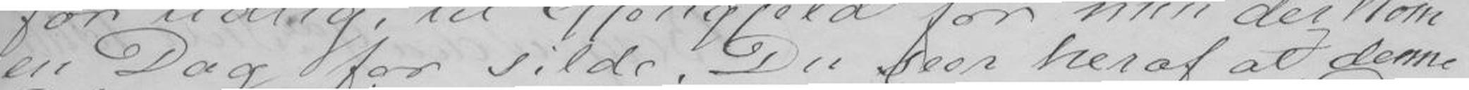en Dag for silde. Du seer heraf at denne
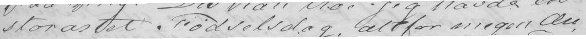storartet Fødselsdag, altfor megen Ære
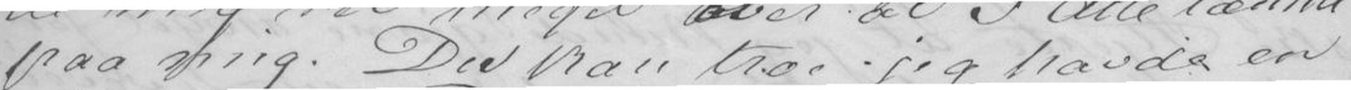paa mig. Der kan troe jeg havde en
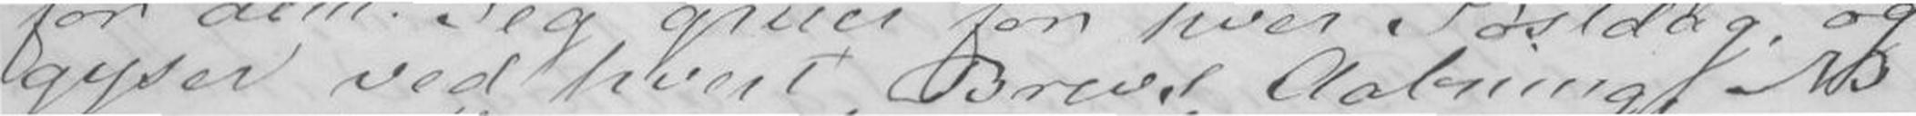gyser ved hvert Brevs Aabning, NB
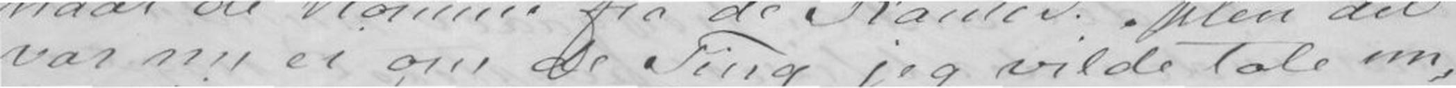var nu ei om de Ting jeg vilde tale nu,
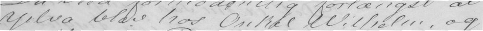Yelva blev hos Onkel Wilhelm, og
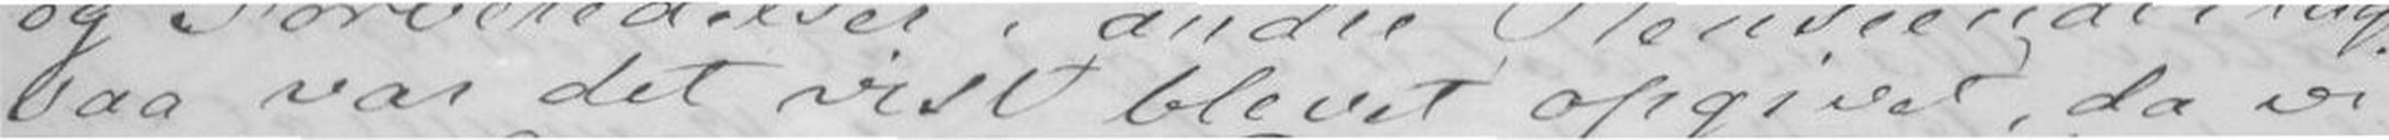saa var det vist blevet opgivet, da vi
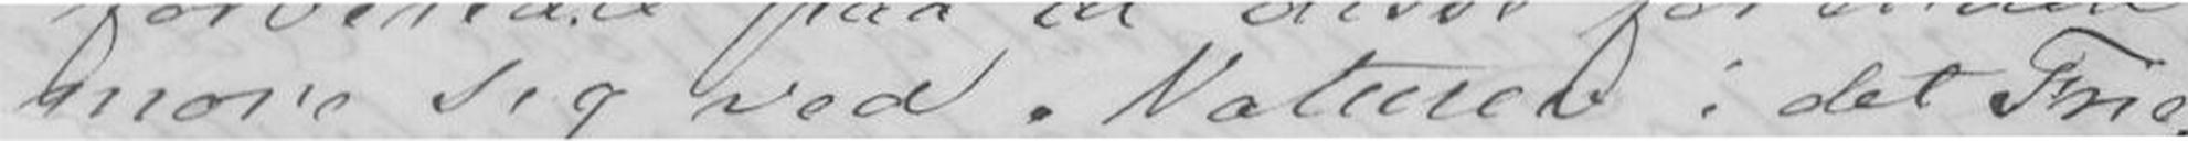more sig ved Naturen i det Frie,
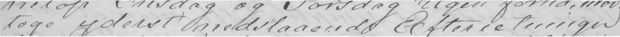tage yderst nedslaaende Efterretninger
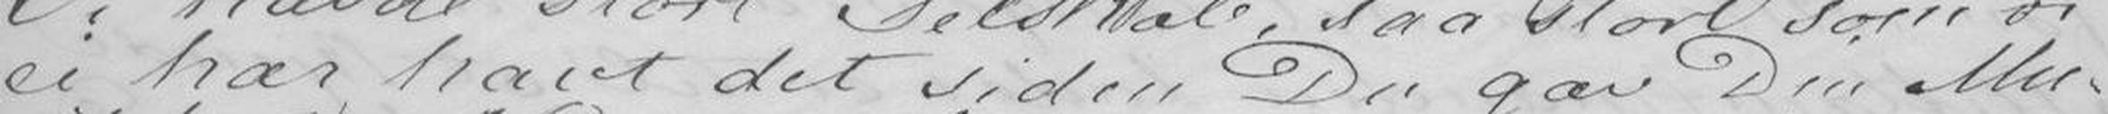ei har havt det siden Du gav Din Mu-
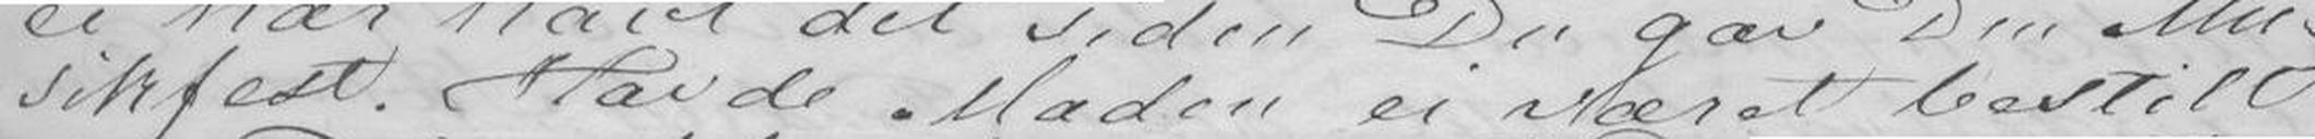sikfest. Havde Maden ei været bestilt
